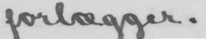forlægger.
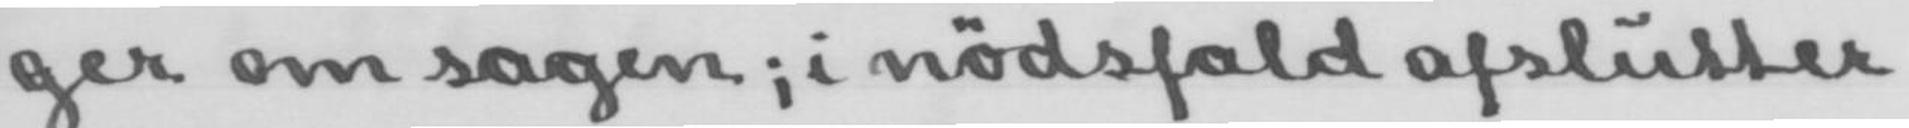ger om sagen; i nødsfald afslutter
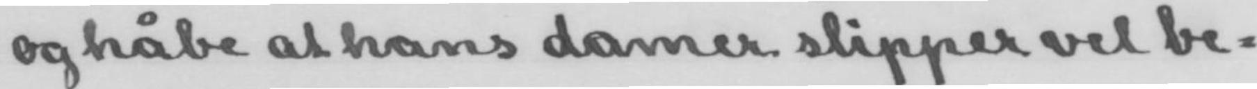og håbe at hans damer slipper vel be-
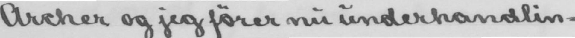Archer og jeg fører nu underhandlin-
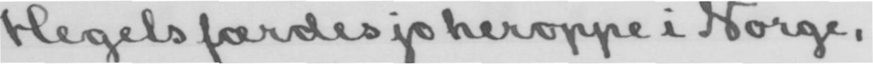Hegels færdes jo heroppe i Norge,
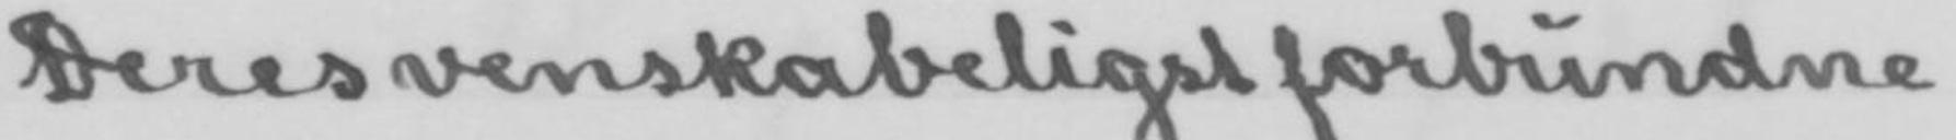Deres venskabeligst forbundne
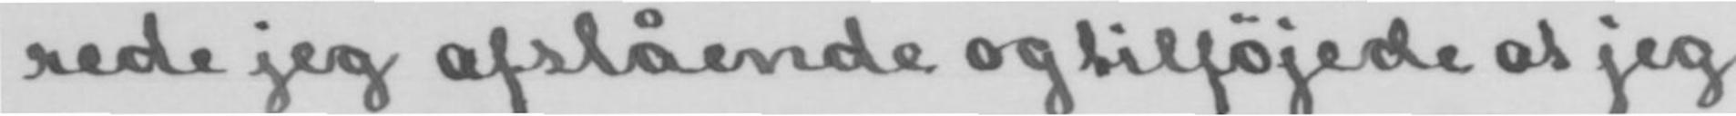rede jeg afslående og tilføjede at jeg
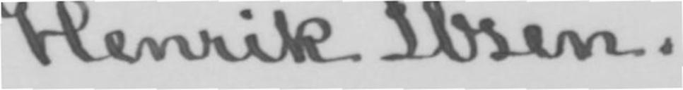Henrik Ibsen.
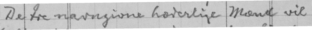De tre navngivne hæderlige Mænd vil
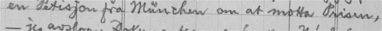en Petisjon fra München om at motta Prisen,
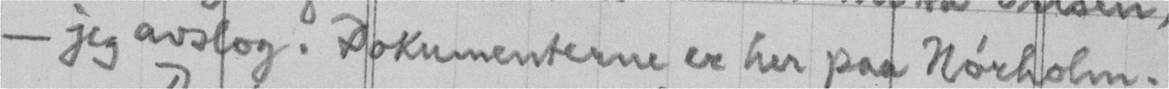- jeg avslog. Dokumenterne er her paa Nørholm.
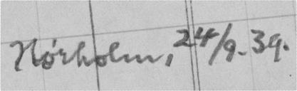Nørholm, 24/9. 39.
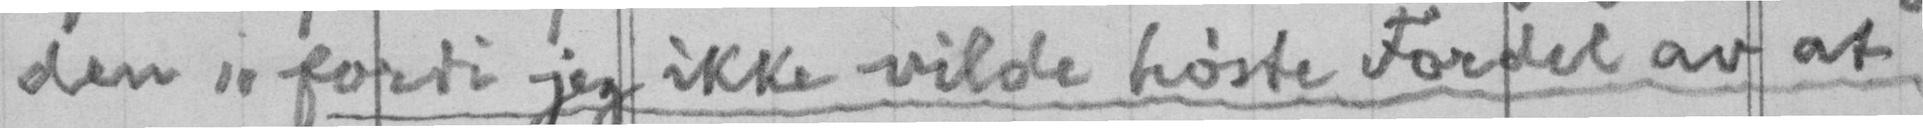den "fordi jeg ikke vilde høste Fordel av at
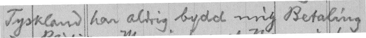Tyskland har aldrig bydd mig Betaling
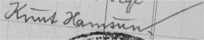Knut Hamsun.
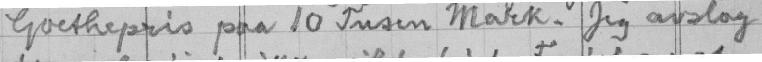Goethepris paa 10 Tusen Mark. Jeg avslog
